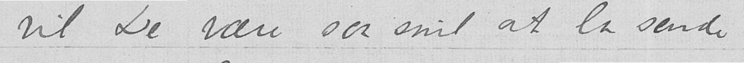vil De være saa snil at la sende
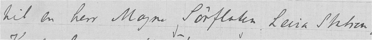til en herr Magne Sørflaten, Leira Station,
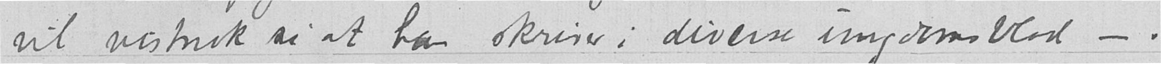vil vistnok si at han skriver i diverse ungdomsblad –.
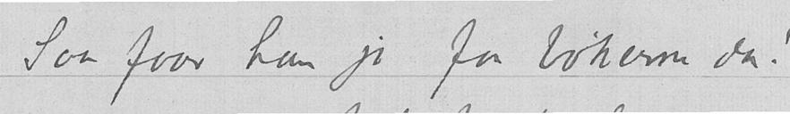Saa faar han jo faa bøkerne da!
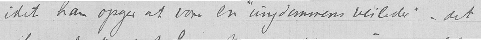idet han opgir at være en "ungdommens veileder" – det
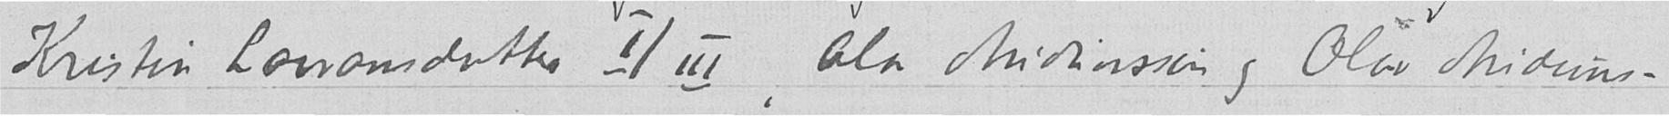Kristin Lavransdatter I/III, Olav Audunssøn og Olav Anduns-
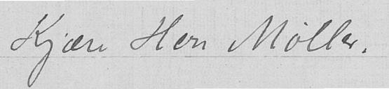Kjære Herr Møller,
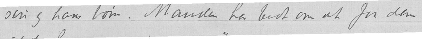søn og hans børn. Manden har bedt om at faa dem
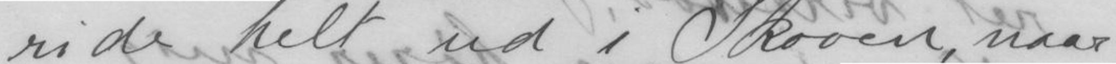ride helt ud i Skoven, naar
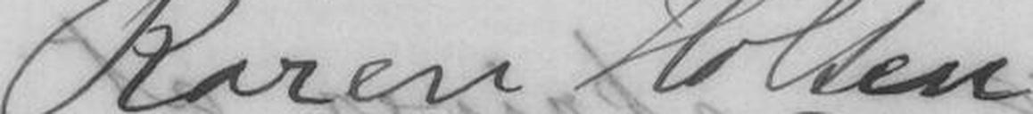Karen Holten
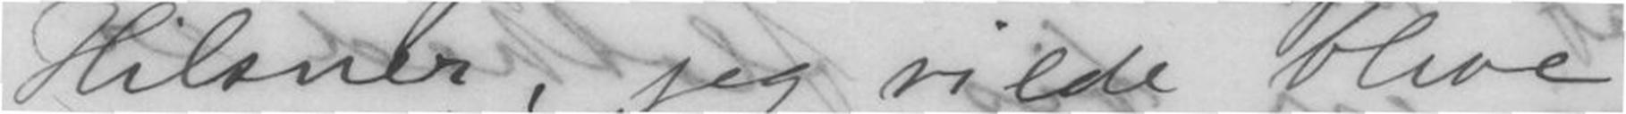Hilsner, jeg vilde blive
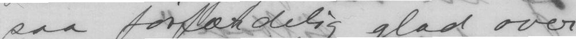saa forfærdelig glad over
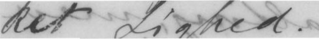ket Lighed.
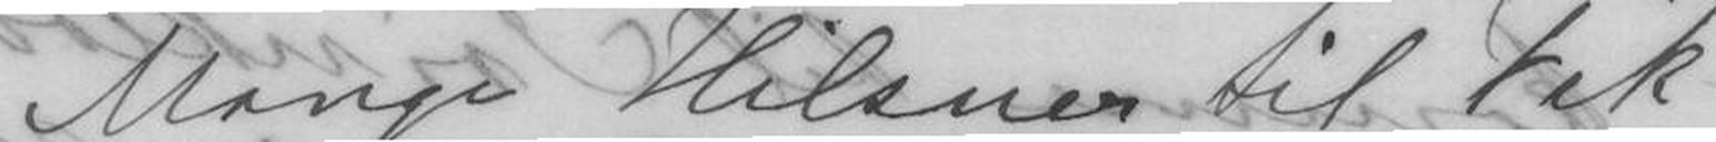Mange Hilsener til Frk.
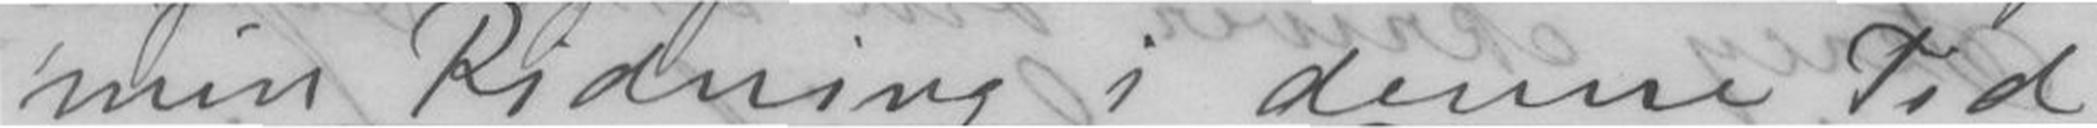min Ridning i denne Tid,
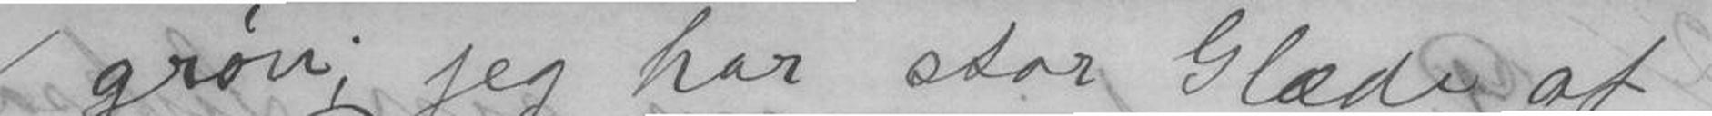grøn, jeg har stor Glæde af
